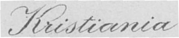Kristiania
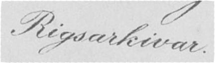Rigsarkivar.
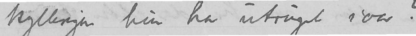kyllingen hun har utruget iaar?
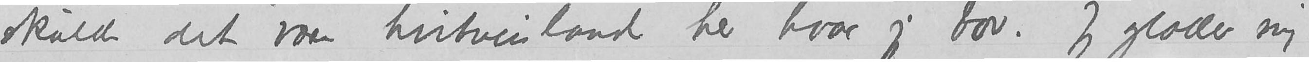skulde det være hvitveisland her hvor jeg bor. Jeg glæder mig
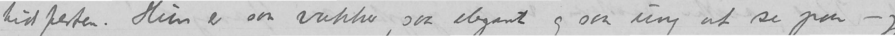tidsfesten. Hun er saa vakker, saa elegant og saa ung at se paa – jeg
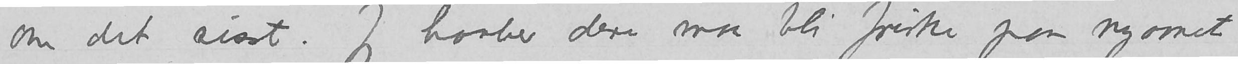om det sisst. Jeg haaber dere maa bli friske paa nyaaret
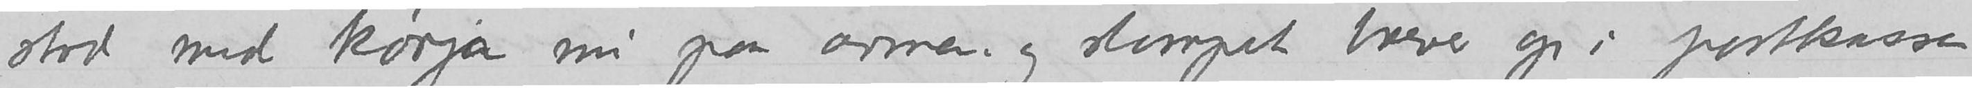stod med kørja mi på armen og stampet brever op i postkassen
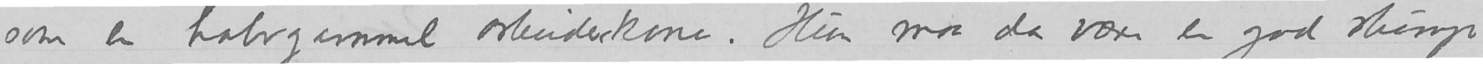som en halvgammel arbeiderkone. Hun maa da være en god slump
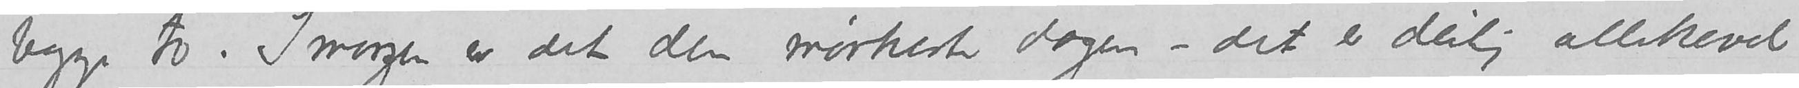begge to. Imorgen er det den mørkeste dagen – det er deilig allikevel
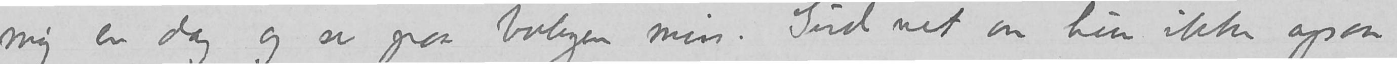mig en dag og se paa babyen min. Gud vet om hun ikke ogsaa
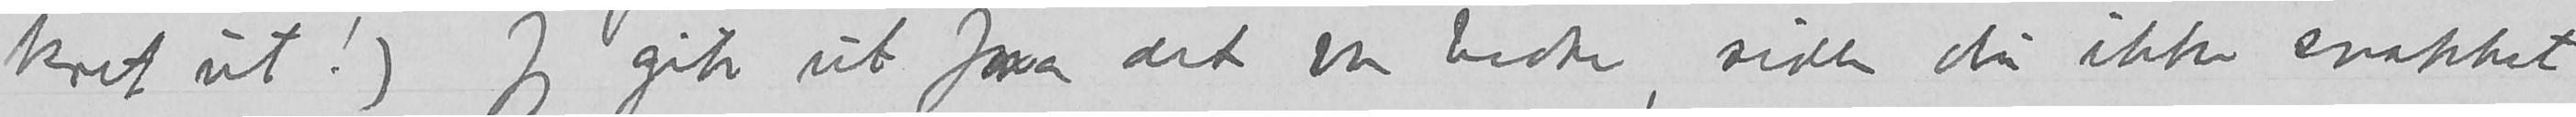skret ut!) Jeg gik ut fra det var bedre, siden du ikke snakket
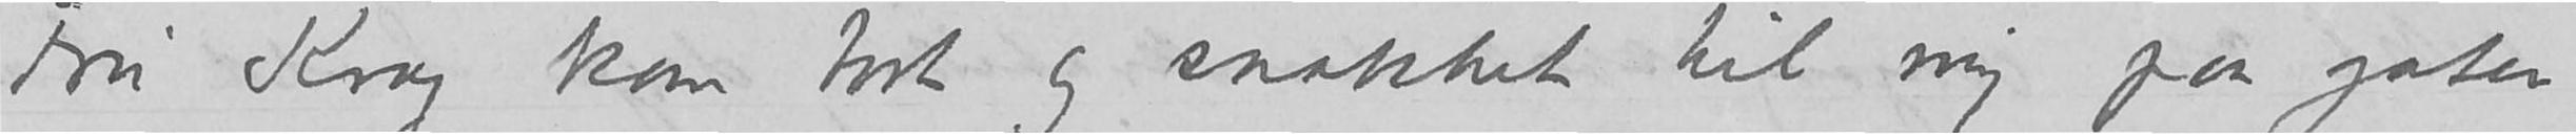Fru Krog kom bort og snakket til mig paa gaten
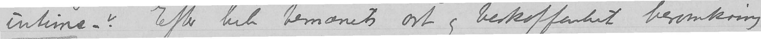intime –? Efter hele bemanets domænets art og beskaffenhet heromkring
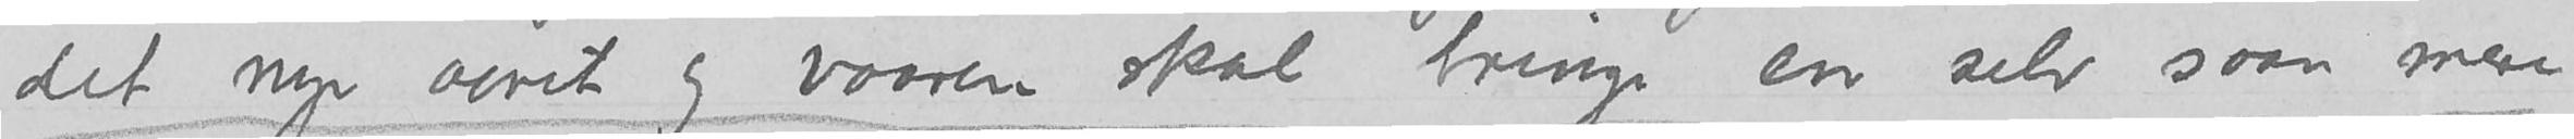det nye aaret og vaaren skal bringe en selv saan mere
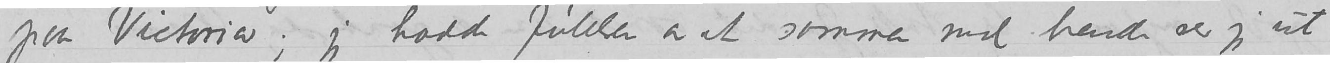paa Victoria; jeg hadde følelsen av at sammen med hende ser jeg ut
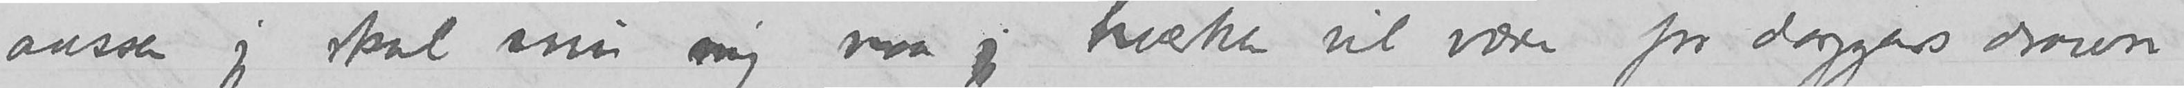aassen jeg skal snu mig, men jeg hverken vil være for daggers drawn
